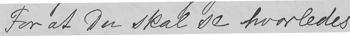For at Du skal se hvorledes
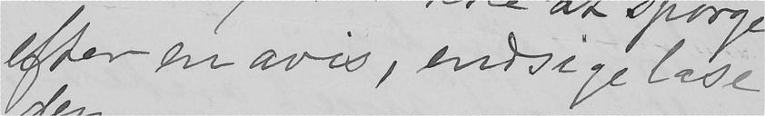efter en avis, endsige læse
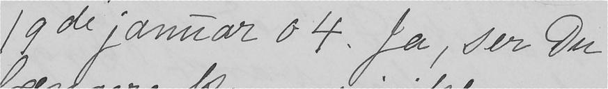19de januar 04. Ja, ser Du
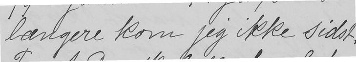længere kom jeg ikke sidst.
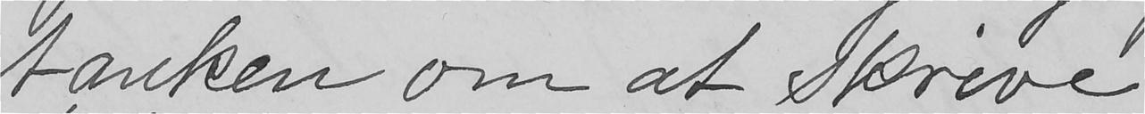tanken om at skrive
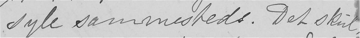syle sammesteds. Det skul-
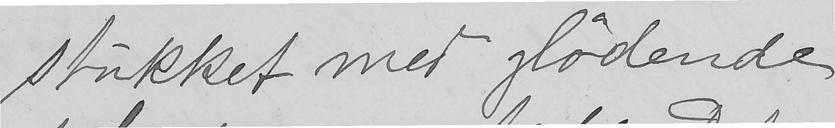stukket med glødende
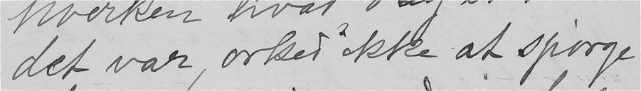det var, orked ikke at spørge
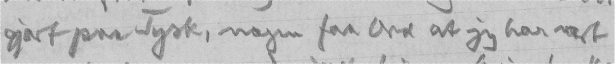gjort paa Tysk, nogen faa Ord at jeg har vært
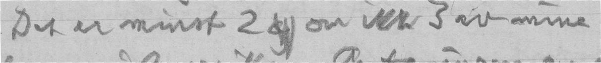Det er minst 2 og om ikke 3 av mine
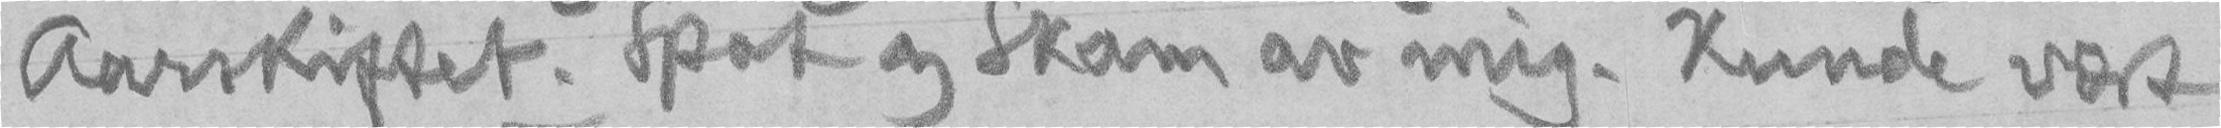Aarskiftet. Spot og Skam av mig. Kunde vært
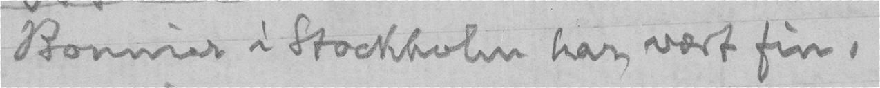Bonnier i Stockholm har vært fin,
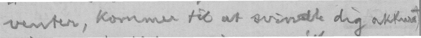venter, kommer til at svindle dig akkurat
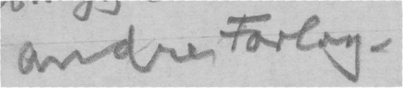andre Forlag.
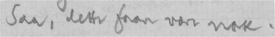Saa, dette faar være nok.
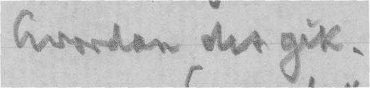hvordan det gik.
